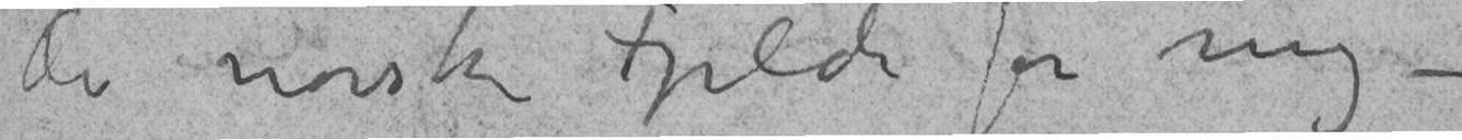de norske Fjelde for mig –
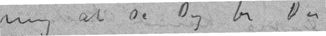mig at se Dig for Du
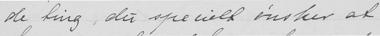de ting, du specielt ønsker at
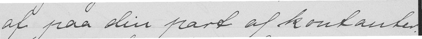af paa din part af kontanter.
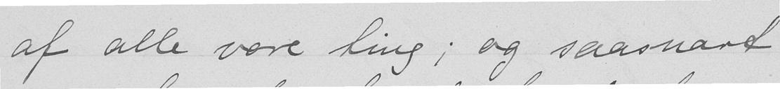af alle vore ting; og saasnart
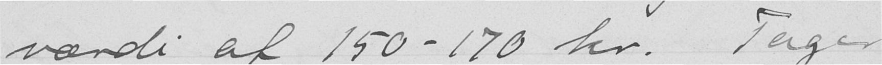værdi af 150-170 kr. Tager
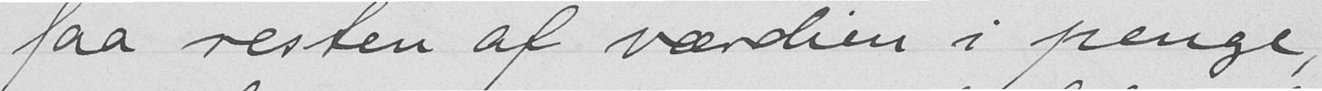faa resten af værdien i penge,
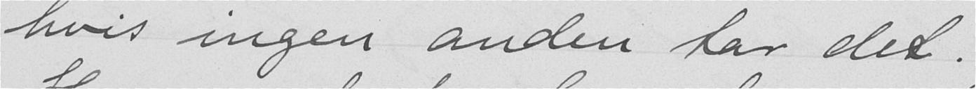hvis ingen anden tar det.
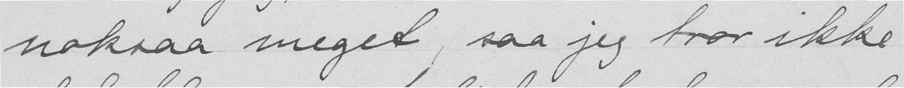noksaa meget, saa jeg tror ikke
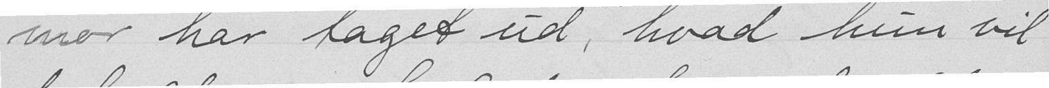mor har taget ud, hvad hun vil
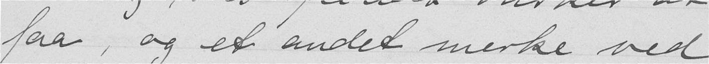faa, og et andet merke ved
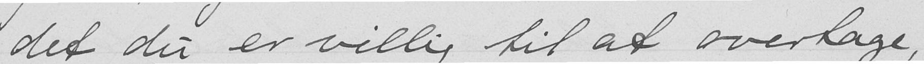det du er villig til at overtage,
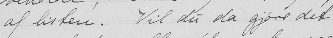af listen. Vil du da gjøre det
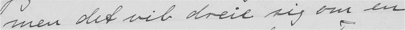men det vil dreie sig om en
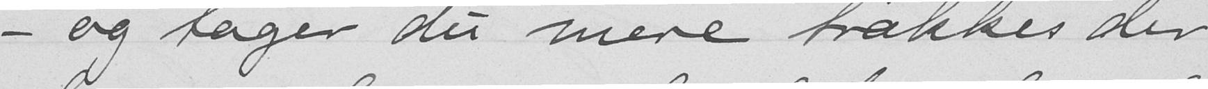- og tager du mere trækkes der
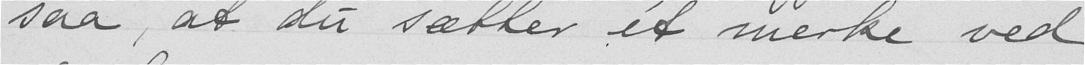saa, at du sætter et merke ved
